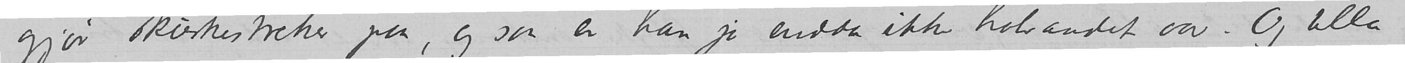gjør skurkestreker paa, og saa er han jo endda ikke halvandet aar. Og Ulla
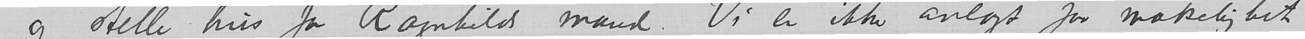og stelle hus for Ragnhilds mand. Vi er ikke anlagt for makelighet
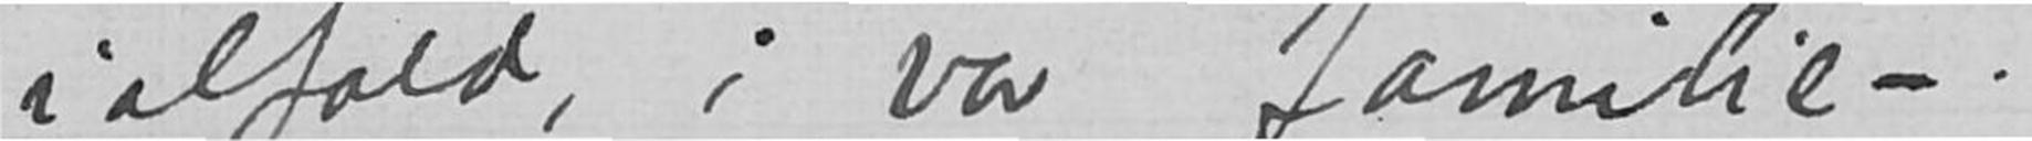ialfald i vor familie –.
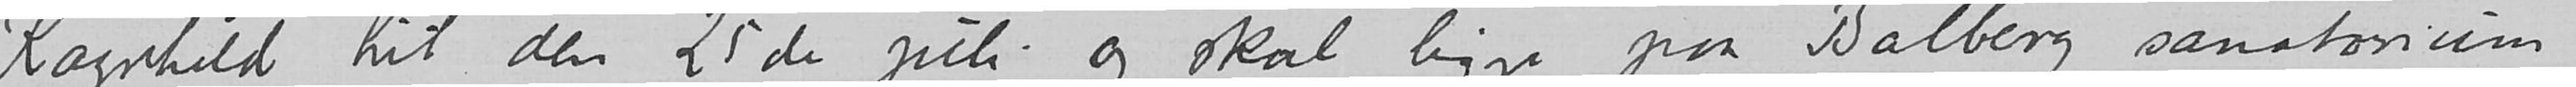Ragnhild hit den 25de juli og skal ligge paa Balberg sanatorium
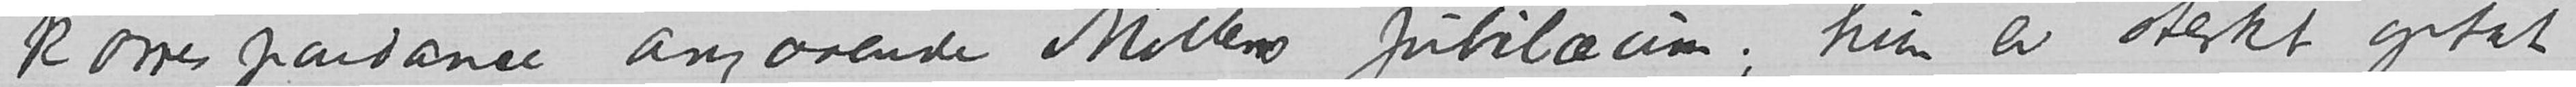korrespondanse angaaende Millens jubilæum; hun er sterkt optat
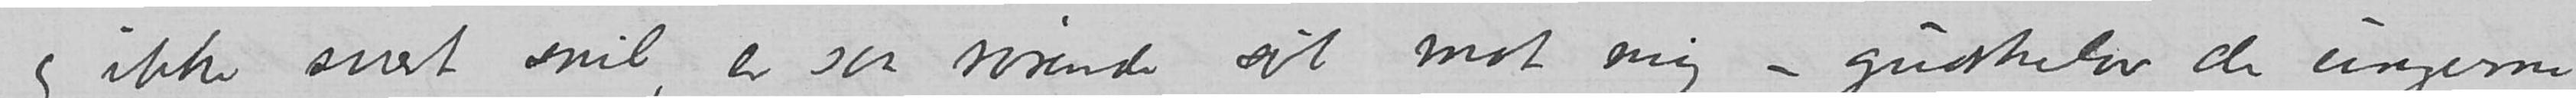og ikke svært snil, er saa rørende søt mot mig- gudskelov de ungerne
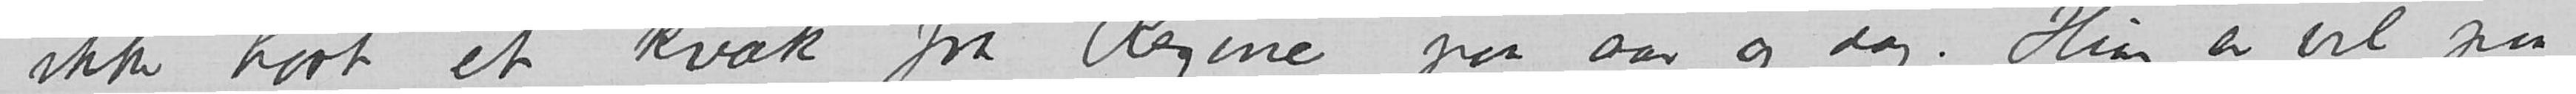ikke hørt et kvæk fra Regine paa aar og dag. Hun er vel paa
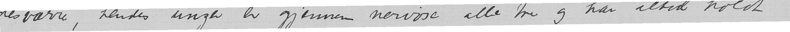desværre, hendes unger er gjennem nervøse alle tre og har altid holdt
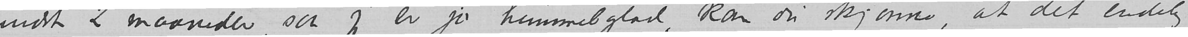mindst 2 maaneder saa jeg er jo himmelglad, kan du skjønne, at det endelig
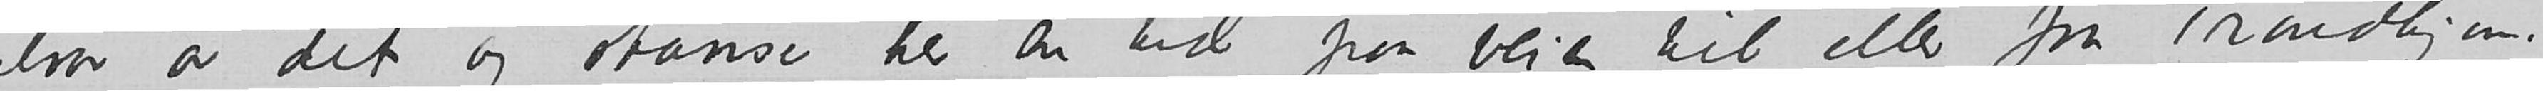alvor av det og stanse her en tid paa veien til eller fra Trondhjem.
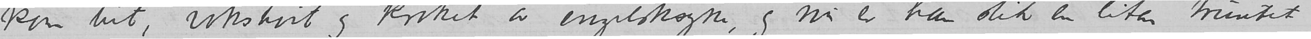kom hit, vokshvit og krøket av engelsksyke, og nu er han slik en liten truntet
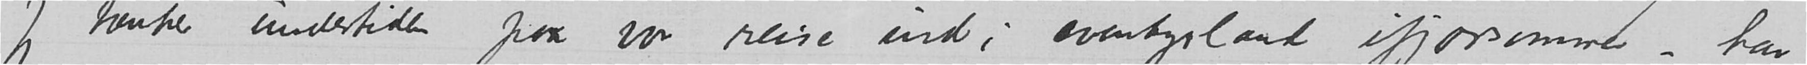Jeg tænker undertiden paa vor reise ind i eventyrland ifjorsommer – har
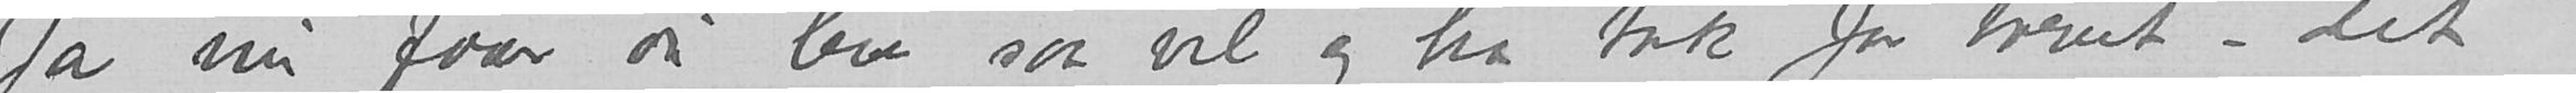Ja nu faar du leve saa vel og ha tak for brevet – det
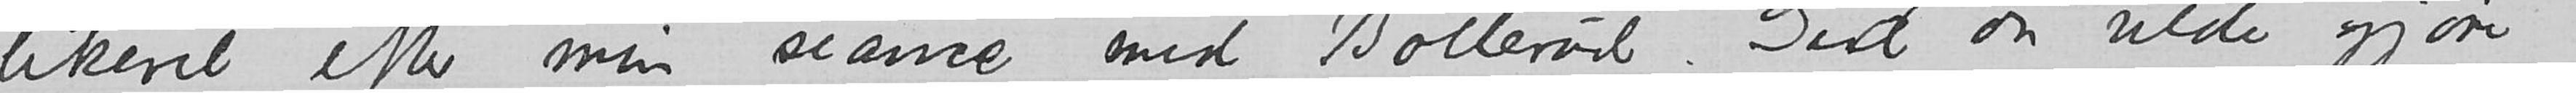likevel efter min seance med Bollerud. Gid du vilde gjøre
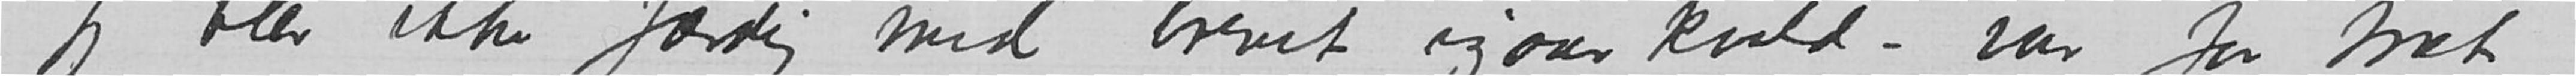Jeg blev ikke færdig med brevet igaar kveld – var for tret
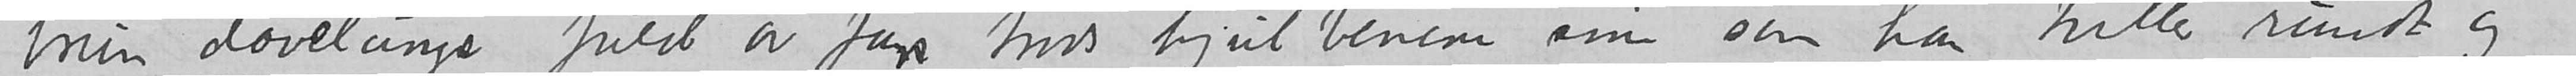brun dævelunge, fuld av <faen> tros hjulbenene sine som han triller rundt og
